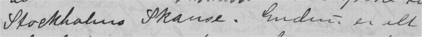Stockholms Skanse. Endnu er alt
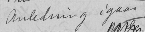Anledning igaar
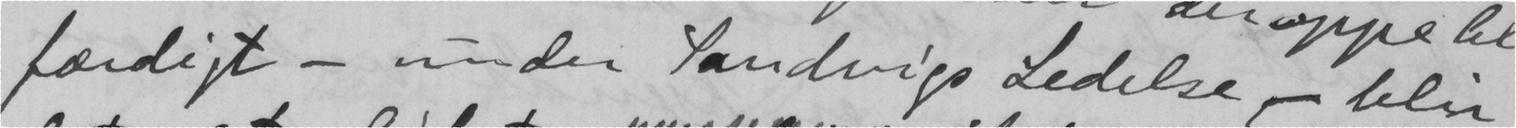færdigt - under Sandvigs Ledelse - blir
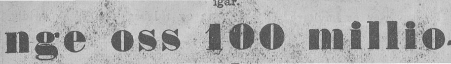nge oss 100 millio-
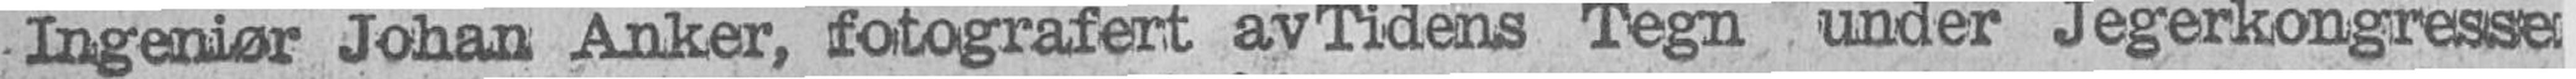Ingeniør Johan Anker, fotografert av Tidens Tegn under Jegerkongressen
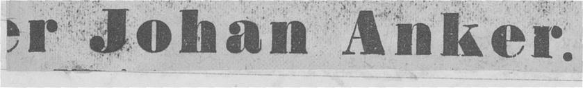er Johan Anker.
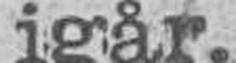igår.
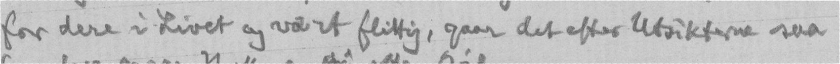for dere i Livet og vært flittig, gaar det efter Utsikterne saa
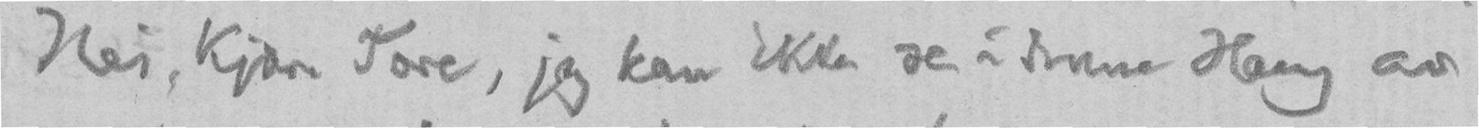Nei, Kjære Tore, jeg kan ikke se i denne Haug av
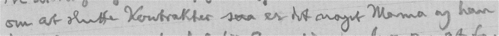om at slutte Kontrakter saa er det noget Mama og han
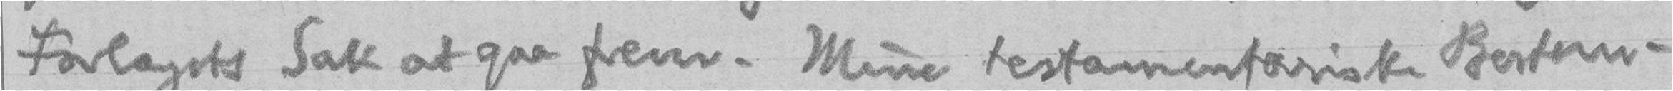Forlagets Sak at gaa frem. Mine testamentariske Bestem-
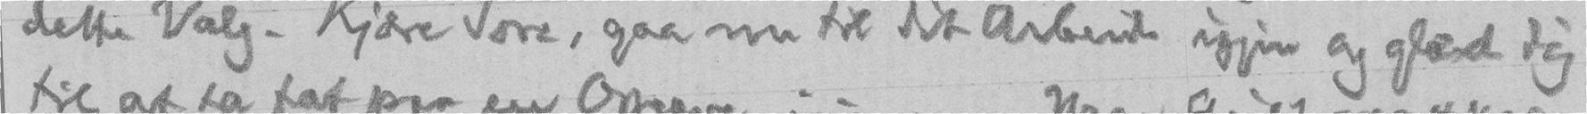dette Valg. Kjære Tore, gaa nu til dit Arbeide igjen og glæd dig
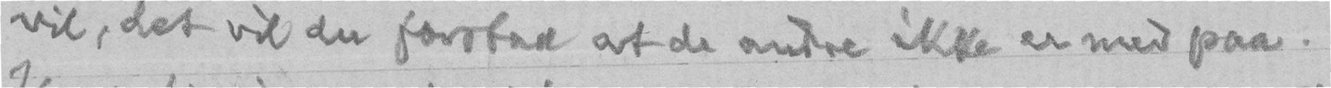vil, det vil du forstaa at de andre ikke er med paa.
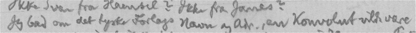Jeg bad om det tyske Forlags Navn og Adr., en Konvolut vilde være
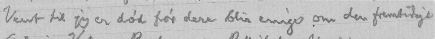Vent til jeg er død før dere blir enige om den fremtidige
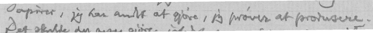Papirer, jeg har andet at gjøre, jeg prøver at produsere.
